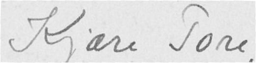Kjære Tore,
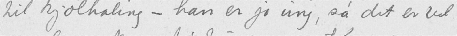til kjølhaling - han er jo ung, så det er vel
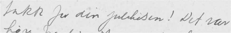takk for din julehilsen! Det var
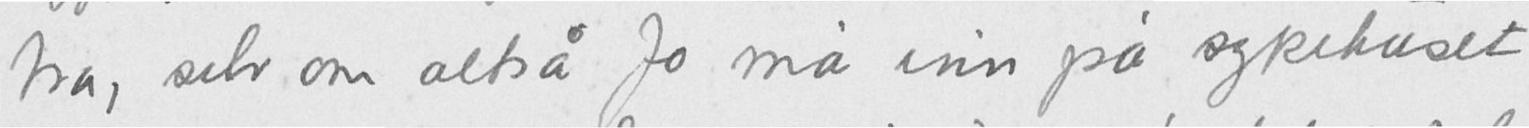bra, selv om altså Jo må inn på sykehuset
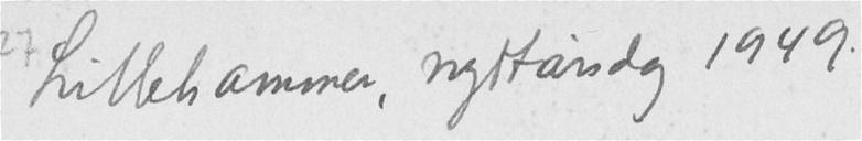Lillehammer, nyttårsdag 1949.
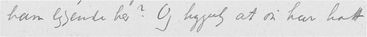ham liggende her? Og hyggelig at du har hatt
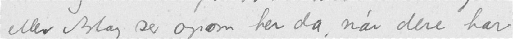eller Åslaug ser opom her da, når dere har
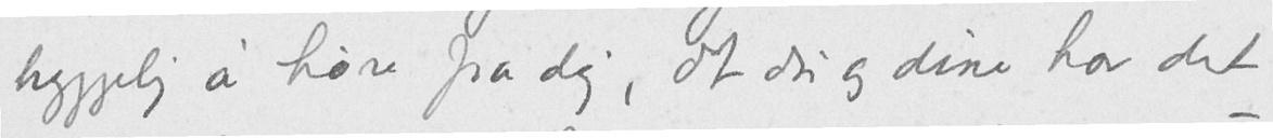hyggelig å høre fra dig, at du og dine har det
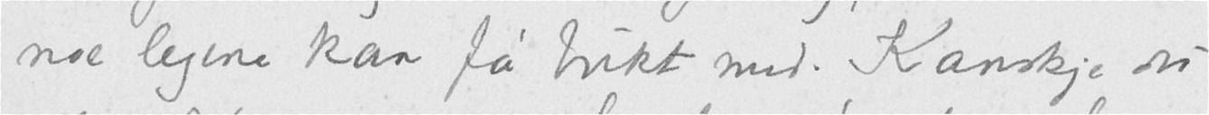noe legene kan få bukt med. Kanskje du
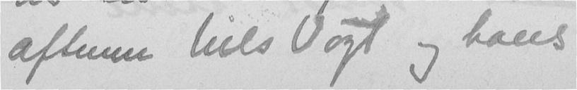aftenen Nils Vogt og hans
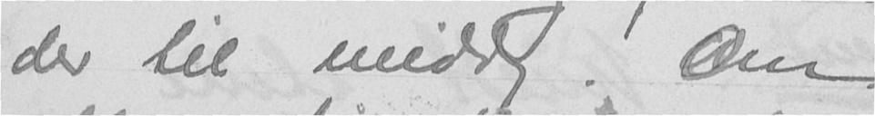der til middag. Om
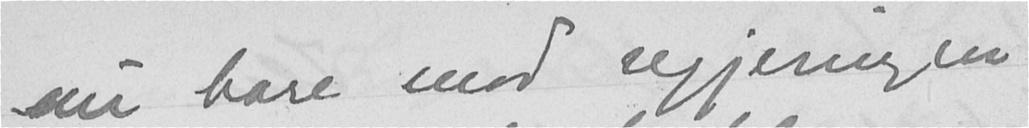nu bare mod regjeringen
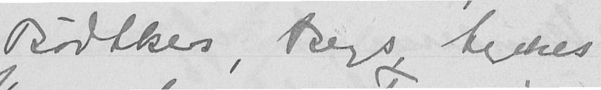Bødtkers, Bergs, Lyches
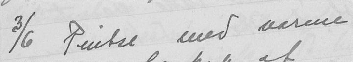3/6 Pintse med varme
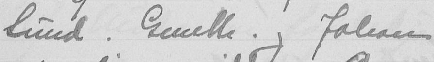Sund. Gunh. og Johan
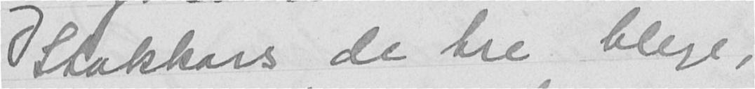Stakkars de tre blege,
